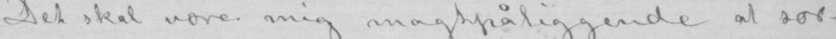Det skal være mig magtpåliggende at sør-
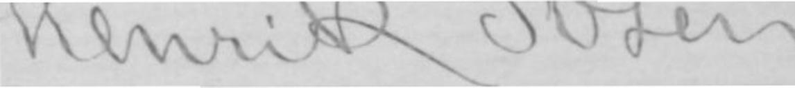Henrik Ibsen.
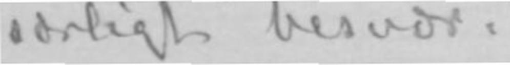særligt besvær.
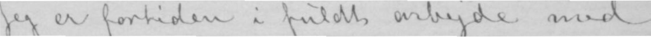Jeg er fortiden i fuldt arbejde med
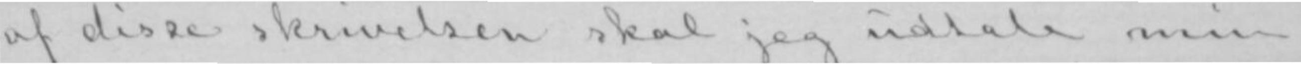af disse skrivelser skal jeg udtale min
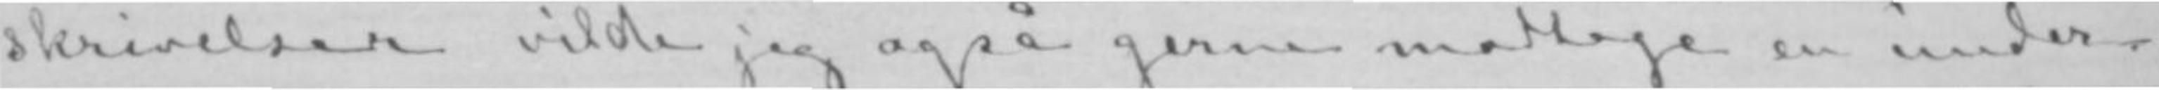skrivelser vilde jeg også gerne modtage en under-
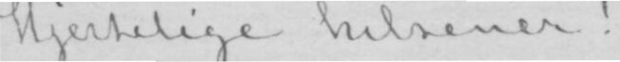Hjertelige hilsener!
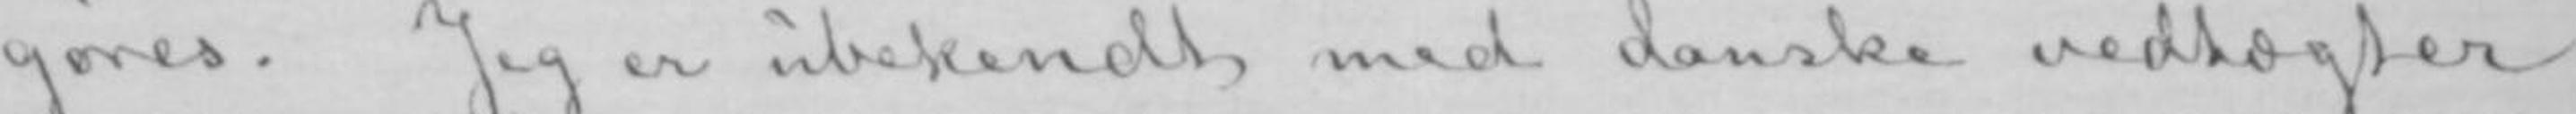gøres. Jeg er ubekendt med danske vedtægter
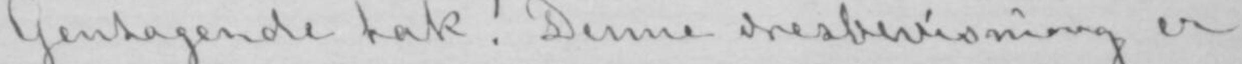Gentagende tak! Denne æresbevisning er
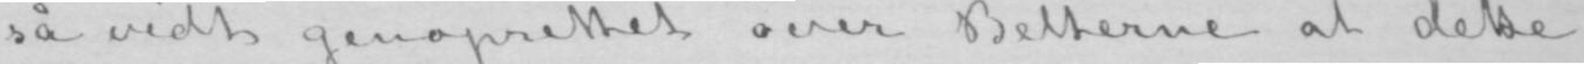så vidt genoprettet over Belterne at dette
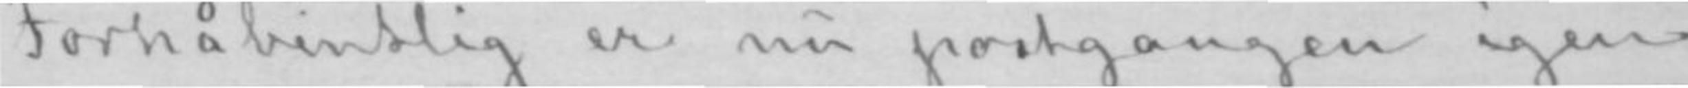Forhåbentlig er nu postgangen igen
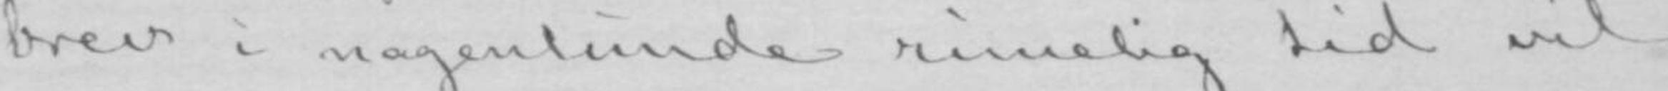brev i nogenlunde rimelig tid vil
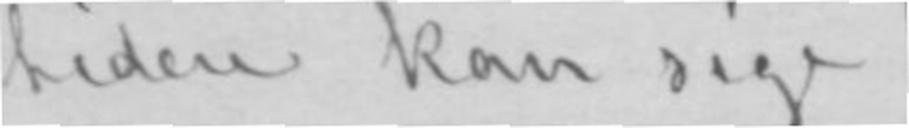tiden kan sige!-
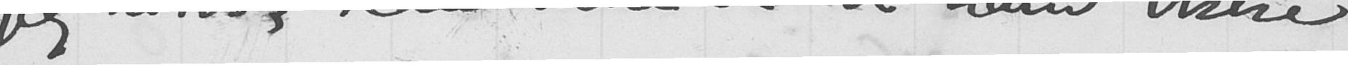jeg hører, kan ikke du be ham ikke
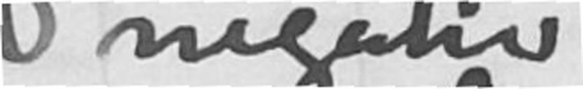negativ
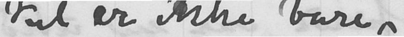kel er ikke bare
